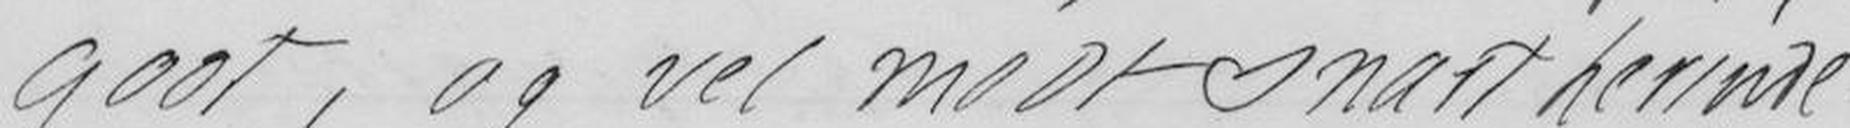godt, og vel mødt snart herinde.
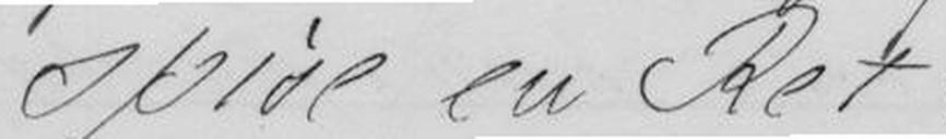spise en Ret
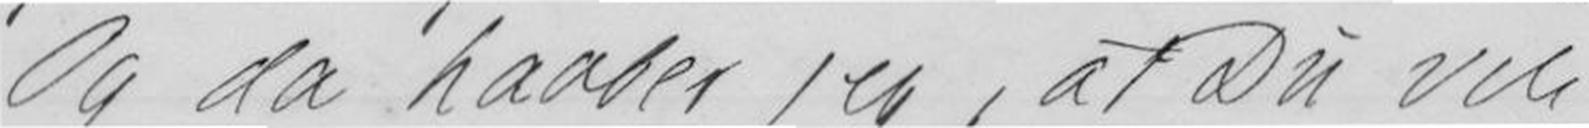Og da haaber jeg, at Du vil
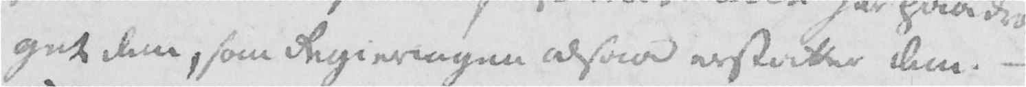get dem, som Regieringen alsaa erstatter dem. -
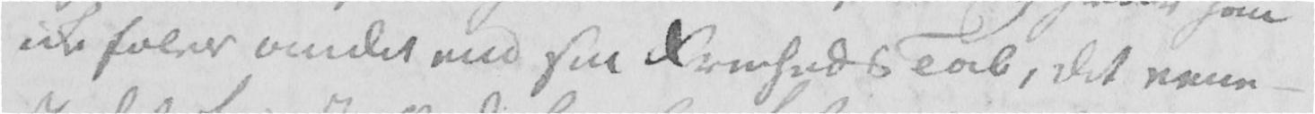icke føler andet end sin Friheds Tab, det eene-
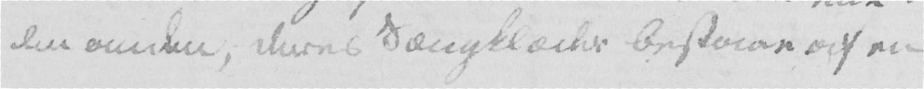den anden, deres Sængklæder bestaae af en
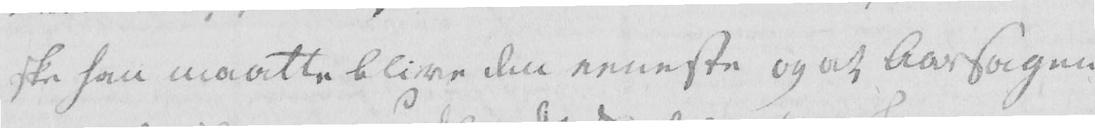ske han maatte blive den eeneste og at Aarsagen
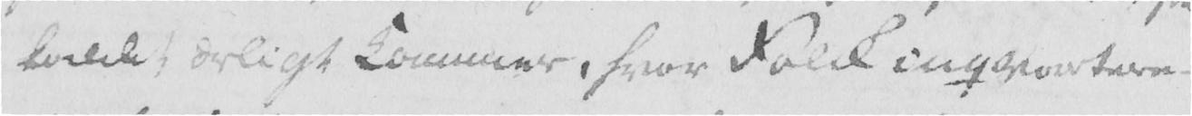kaldet ærligt Kammer, hvor folk inqvartere-
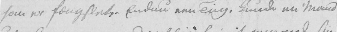han er fængslet. Endnu een Ting. Kunde en Mand
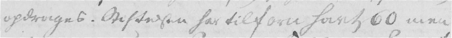opdrages. Stiftelsen har tilforn havt 60 men
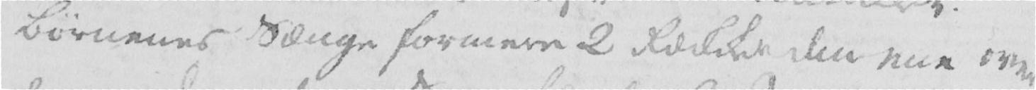Børnenes Sænge formere 2 Rækker den ene over
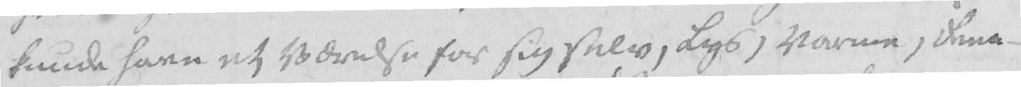kunde have et Værelse for sig selv, Lys, Varme, Ren-
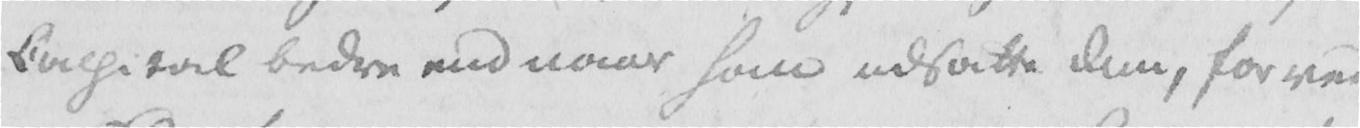Capital bedre end naar han udsatte dem, for ved
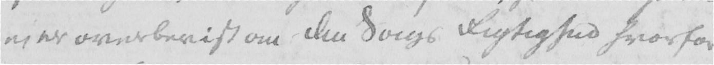ej er overbevist om den Sorgs Rigtighed hvorfor
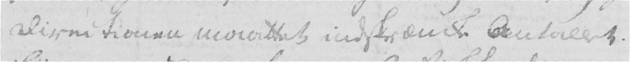Directionen maattet indskrænke Antallet.
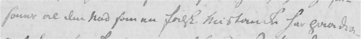soner al den nød som en falsk Mistanke har paadra-
